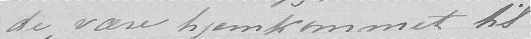de være hjemkommet til
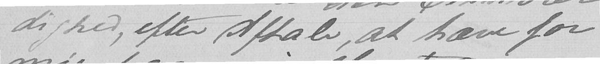dighed, efter Aftale, at hæve for-
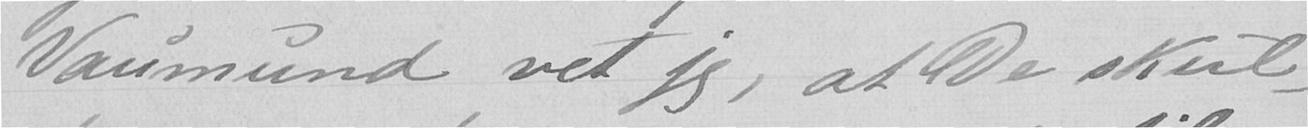Vaumund vet jeg, at De skul-
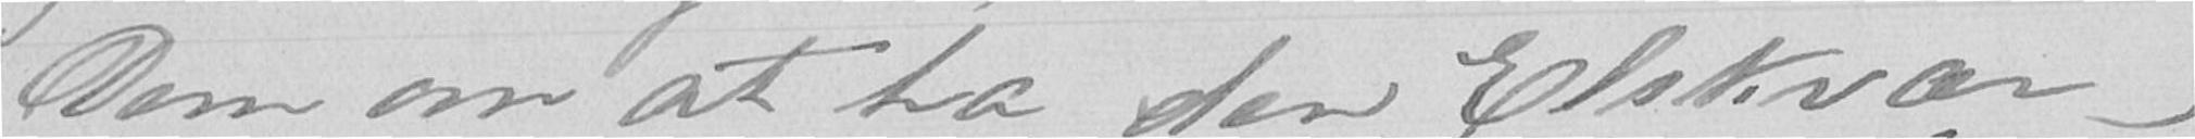Dem om at ha den Elskvær-
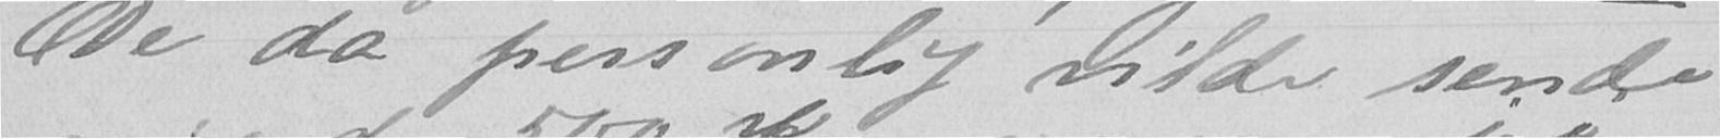De da personlig vilde sende
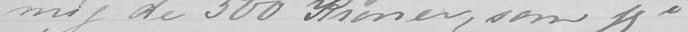mig de 500 Kroner, som jeg i
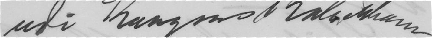udi Kongens København
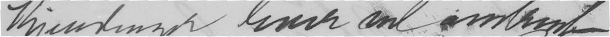Kjendinger lever omtrent
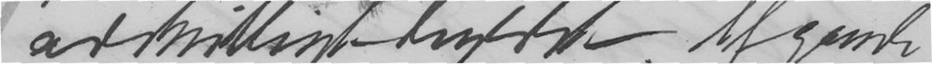adskilligt hyldet. Af gamle
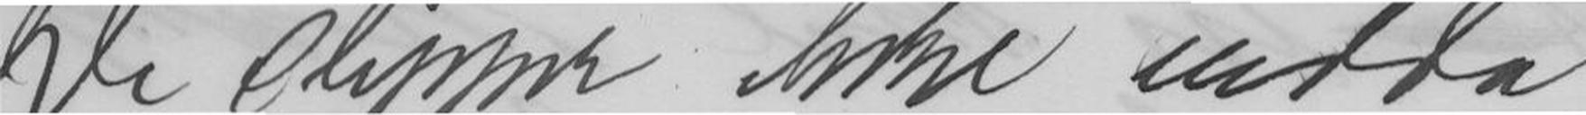De slipper ikke endda.
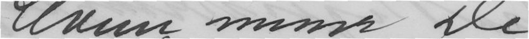Hvem mener De
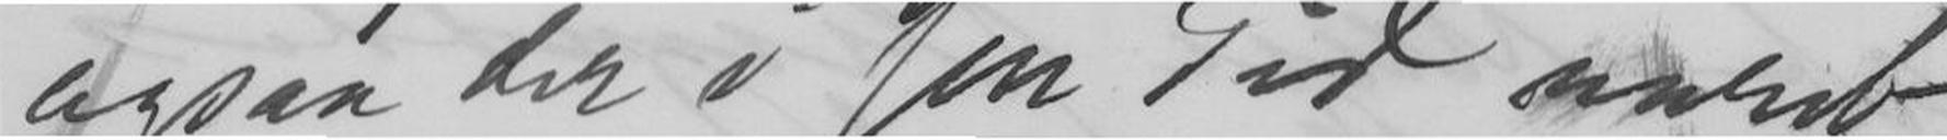ogsaa der i sin Tid været
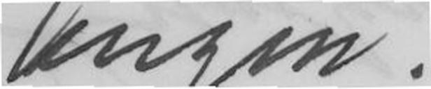ingen.
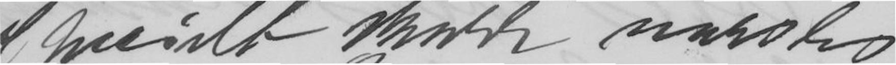spesielt skulde varsles
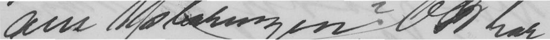om Afsløringen? O. B. har
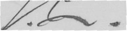J. L.
